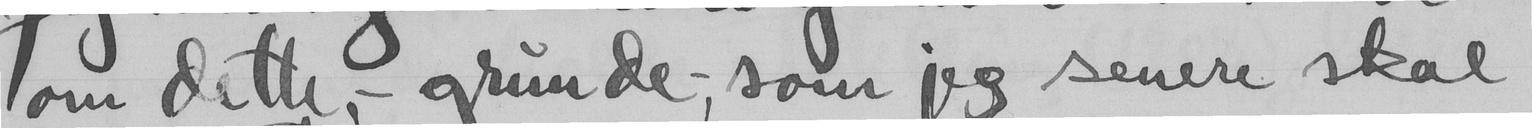om dette, - grunde, som jeg senere skal
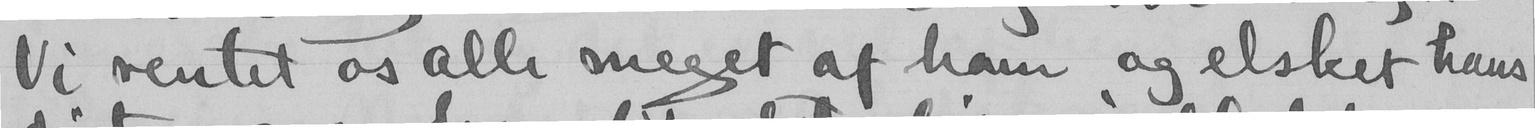Vi ventet os alle meget af ham og elsket hans
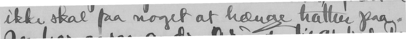ikke skal faa noget at hænge hatter paa.
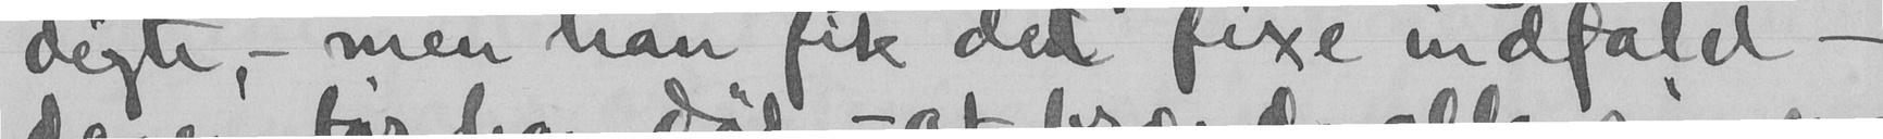digte, - men han fik det fixe indfald -
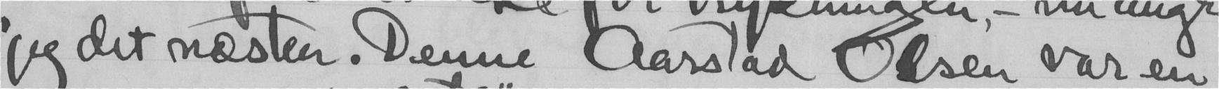jeg det næsten. Denne Aarstad Olsen var en
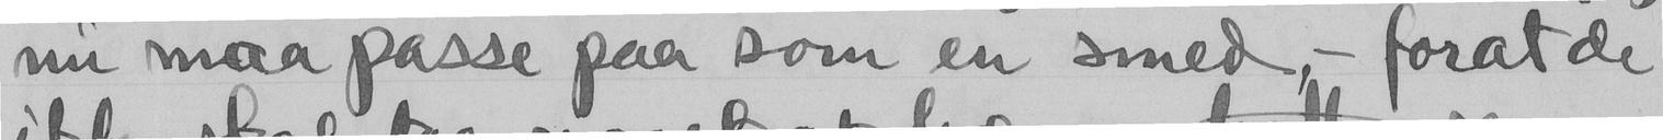nu maa passe paa som en smed, - forat de
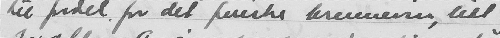til fordel for det finske kremmerier litt
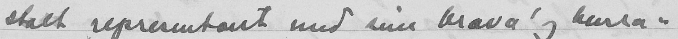stolt representant med sine bravo og hurra-
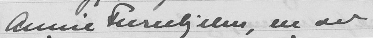Annee Furuhjelm, en av
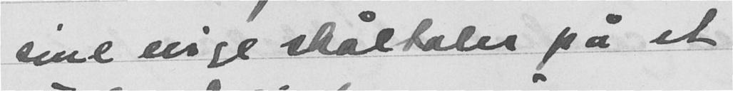sine evige skåltaler pa et
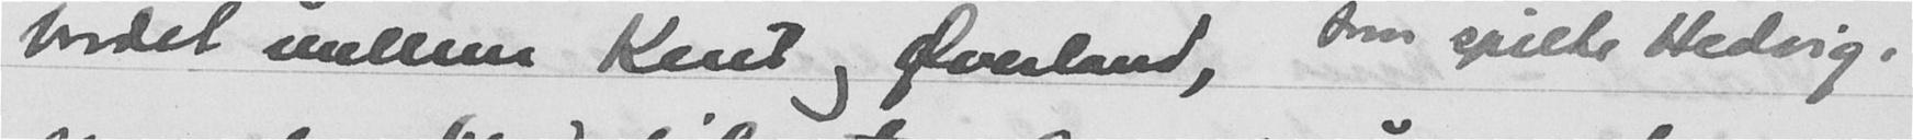bordet mellem Kent og Øverland, som spilte Hedvig.
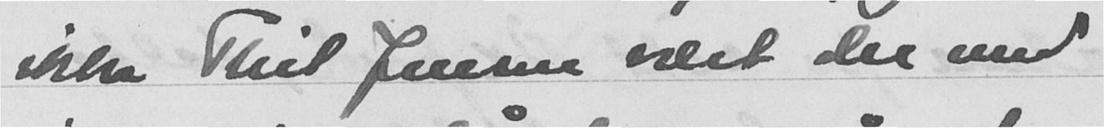ikke Thil Jensen vært der med
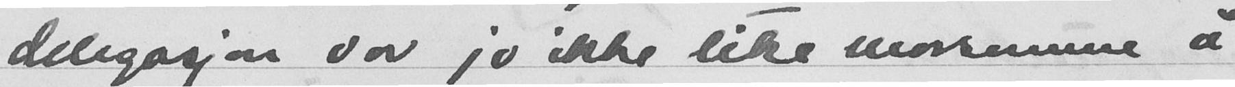delegasjon var jo ikke like morsomme å
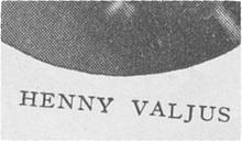Henny Valjus
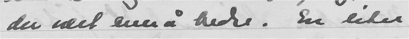der vært ennå bedre. En liten
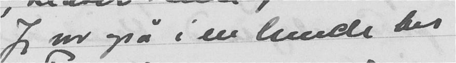Jeg var også i en lunch hos
